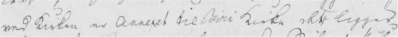ved Kirken er Annexet til Biri Kirke det ligger
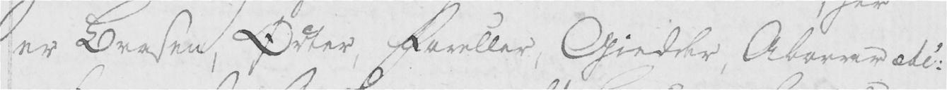er Brasen, Ørter, Fareller, Giedder, Aborrer etc.
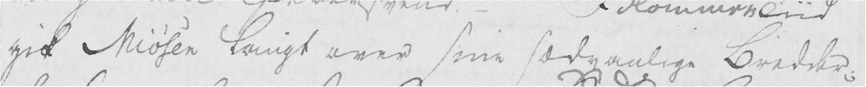gik Miøsen langt over sine sædvanlige Bredder;
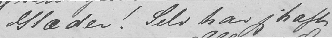Glæder! Selv har jeg haft
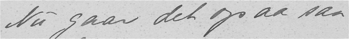Nu gaar det ogsaa saa
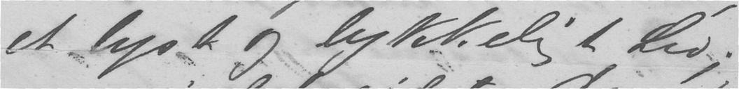et lyst og lykkeligt Liv,
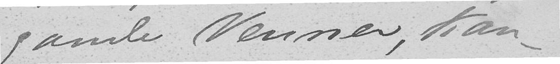gamle Venner, kan-
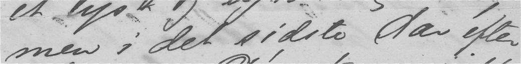men i det sidste Aar efter
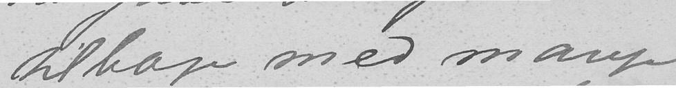tilbage med mange
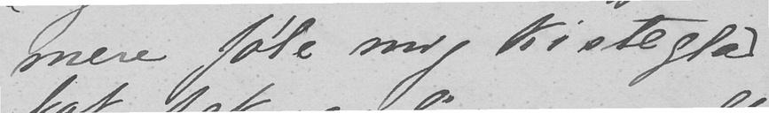mere føle mig kisteglad
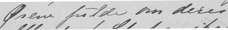Ørene fulde om deres
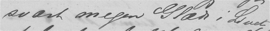svært megen Glæde i Livet,
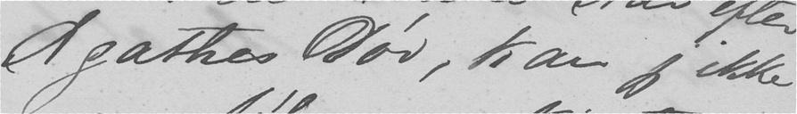Agathes Død, kan jeg ikke
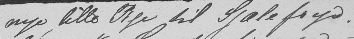nye lille Pige til Sjælefryd.
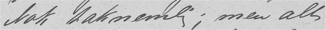Nok taknemlig; men alt
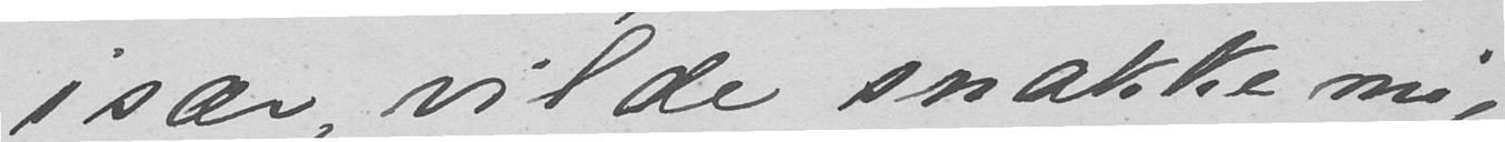især, vilde snakke mig
